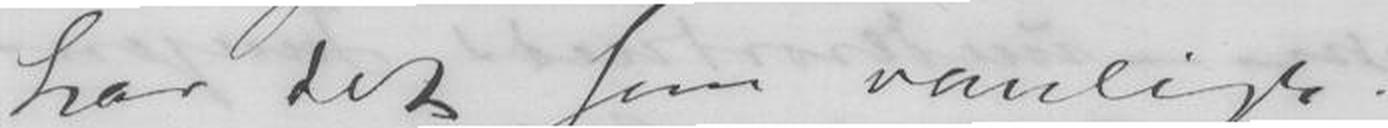har det som vanligt. -
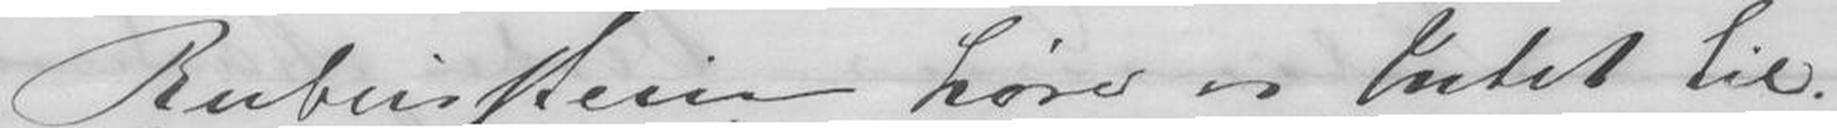Rubinstein hører vi Intet til.
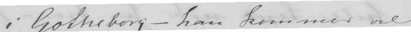i Gotheborg - han kommer vel
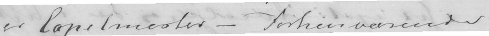er Capelmester - Forhenværende
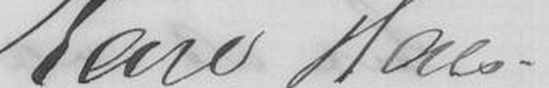Karl Hals.
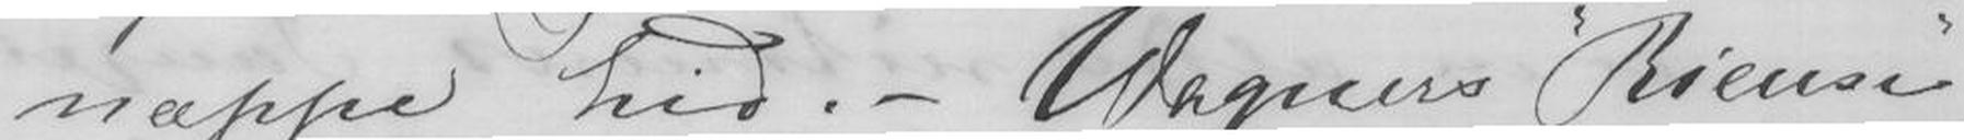næppe hid. - Wagners "Riense"
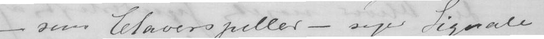- som Claverspiller - siger Signale -
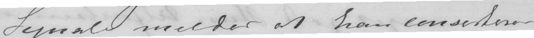Signale melder at han conserterer
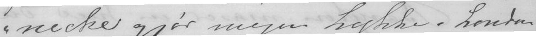necke gjør megen Lykke i London
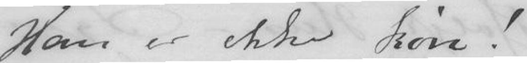Han er ikke køn!
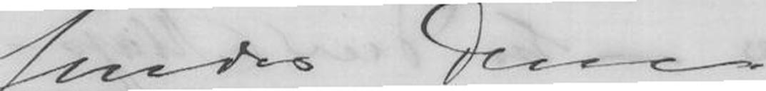sendes Dem
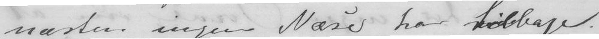næsten ingen Næse har tilbage
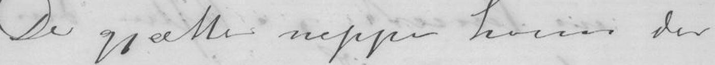De gjætter neppe hvem der
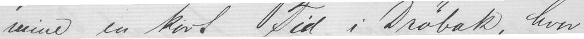mine en kort Tid i Drøbak, hvor
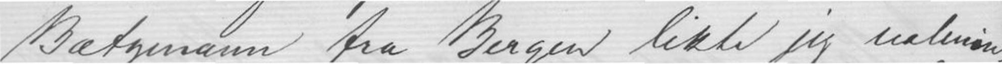Batzmann fra Bergen likte jeg ualmin-
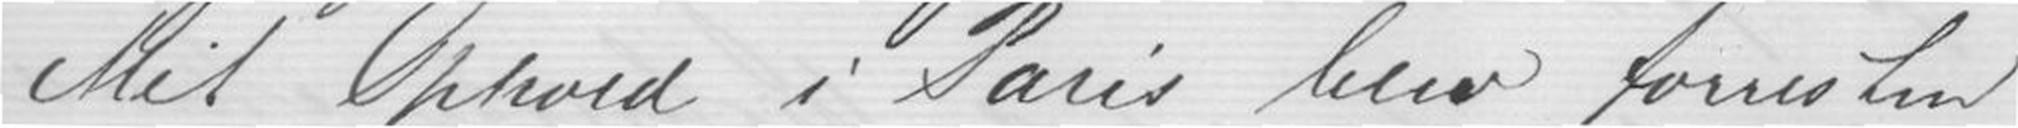Mit Ophold i Paris blev forresten
